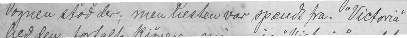Vognen stod der; men hesten var spændt fra. "Victoria"
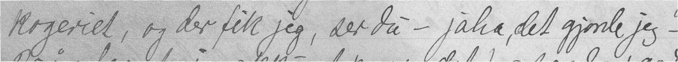kogeriet, og der fik jeg, ser du - jaha, det gjorde jeg -
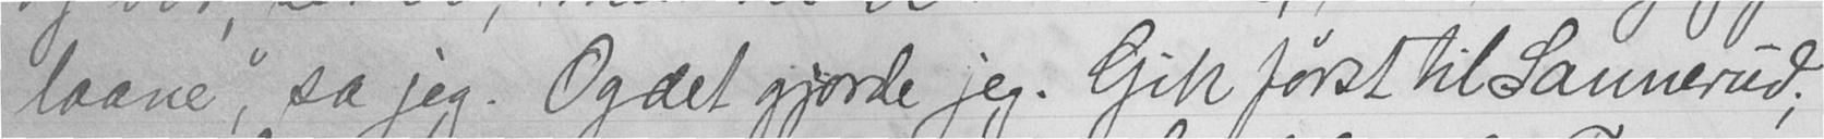laane," sa jeg. Og det gjorde jeg. Gik først til Sannerud;
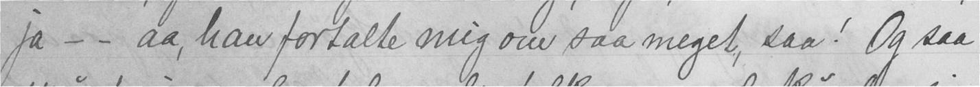ja - - aa, han fortalte mig om saa meget, saa! Og saa
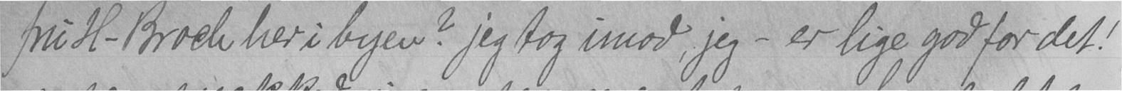fru H - Broch her i byen? jeg tog imod, jeg - er lige god for det!
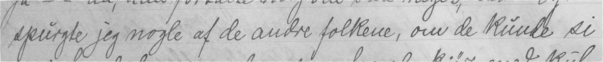spurgte jeg nogle af de andre folkene, om de kunde si
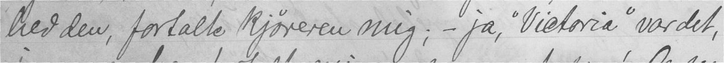hed den, fortalte kjøreren mig; - ja, "Victoria" var det,
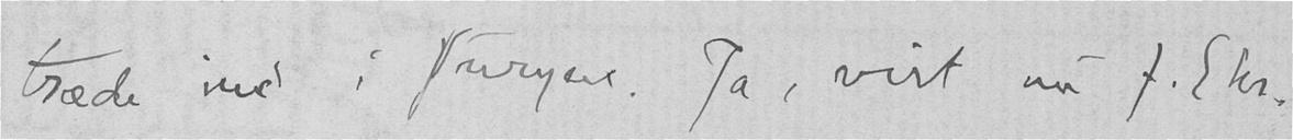træde ind i Juryen. Ja, vist nu f. Eks.
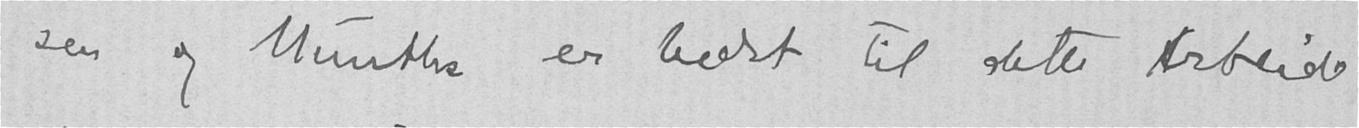sen og Munthe er bedst til dette Arbeide
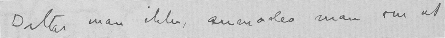Deltar man ikke, anmodes man om at
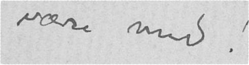være med!
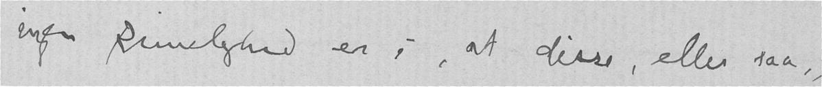ingen Rimelighed er i, at disse, eller saa-
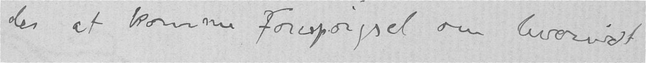der at komme Forespørgsel om hvorvidt
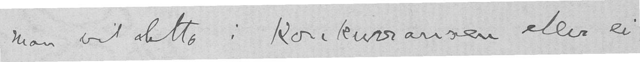man vil delta i Konkurransen eller ei.
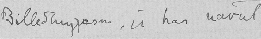Billedhuggere, jeg har nævnt
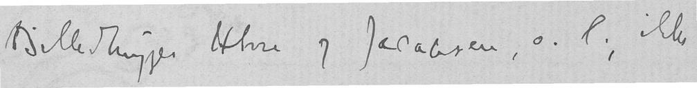Billedhugger Ihre og Jarabsen, o. l. ikke
